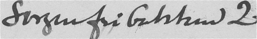Sorgenfribakken 2
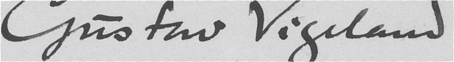Gustav Vigeland
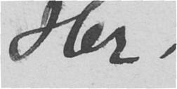Her.
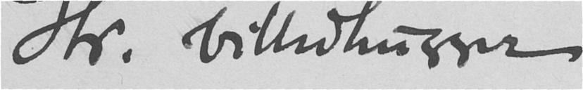Hr. billedhugger
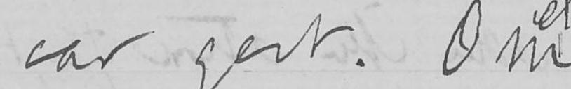aar godt. Om
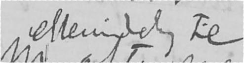eftermiddag til
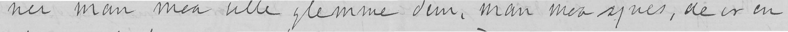nei man maa ville glemme dem, man maa synes, de er en
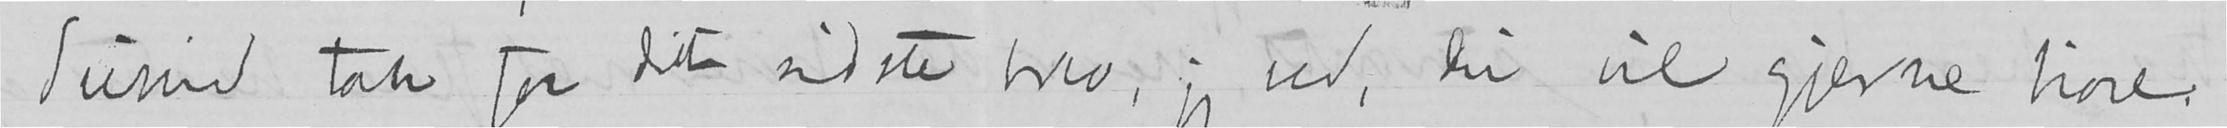Tusind tak for dit sidste brev, jeg ved, Du vil gjerne høre
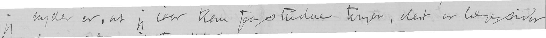jeg nyder er, at jeg iaar kan faa studere tingen, det er længe siden
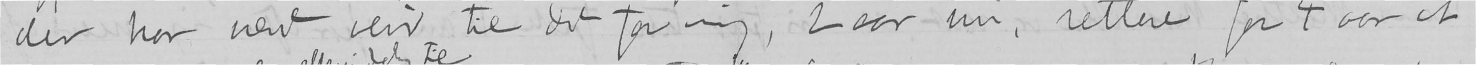der har været veir til det for mig, 2 aar nu, rettere for 4 aar et
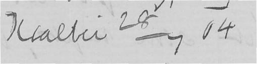Kvalbei 28/7 04
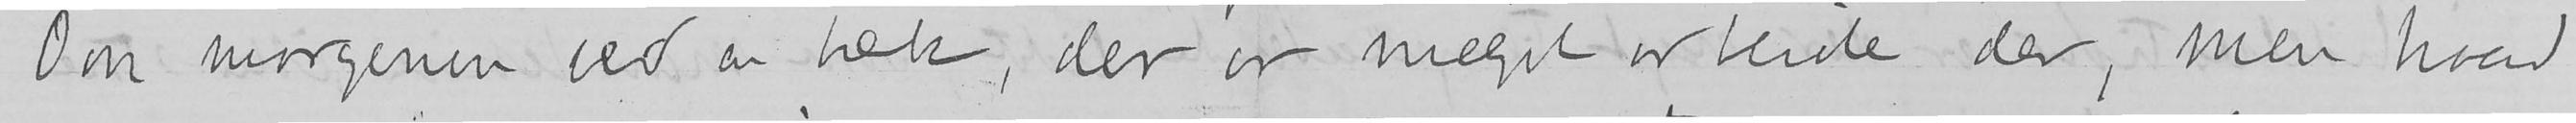Om morgenen ved en bæk, der er meget arbeide der, men hvad
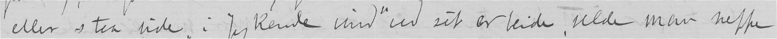eller staa ude "i fykende vind" ved sit arbeide, vilde man neppe
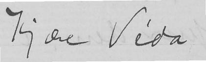Kjære Véda
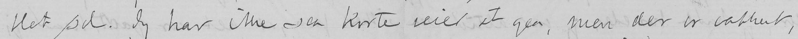blot sol. Jeg har ikke saa korte veier at gaa, men der er vakkert,
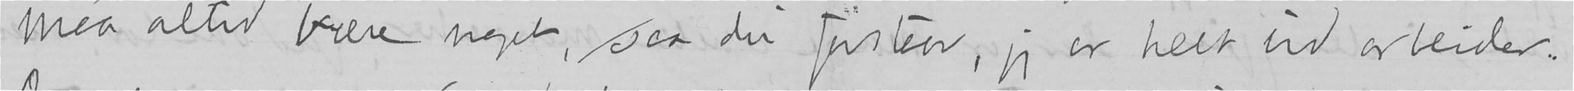maa altid bære noget, saa du forstaar, jeg er helt ud arbeider.
